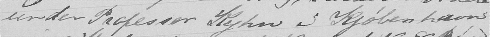under Professor Kyhn i Kjøbenhavn
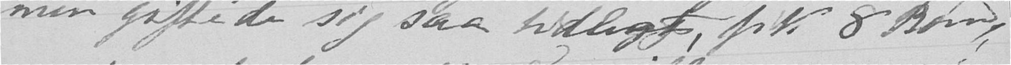men giftede sig saa tidligt, fik 8 Børn,
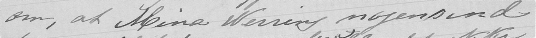om, at Mina Werring nogensinde
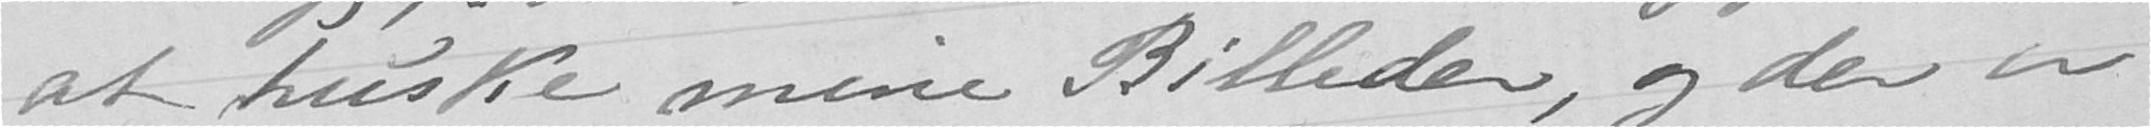at huske mine Billeder, og der er
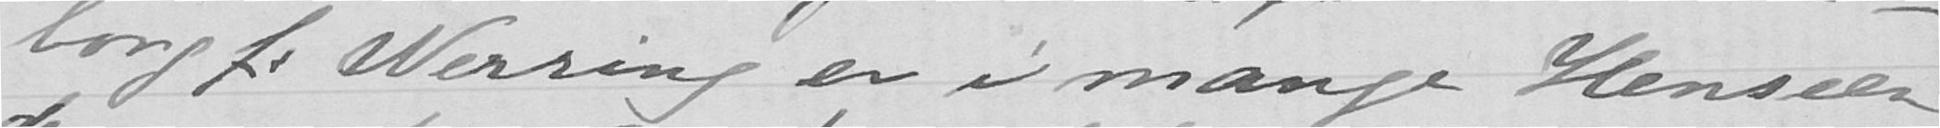borg f. Werring er i mange Henseen-
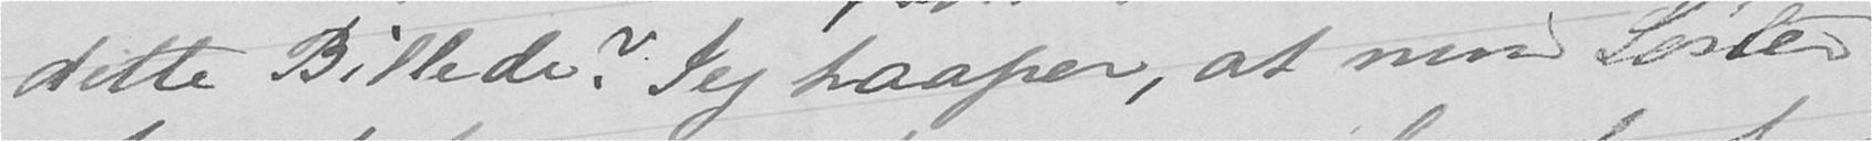dette Billede? Jeg haaper, at min Søster
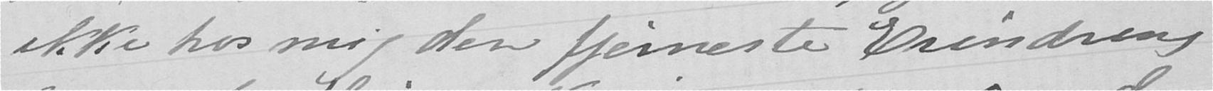ikke hos mig den fjerneste Erindring
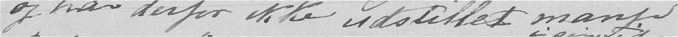og har derfor ikke udstillet mange
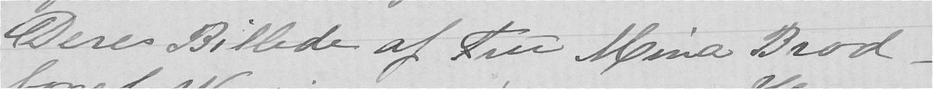Deres Billede af Fru Mina Brod-
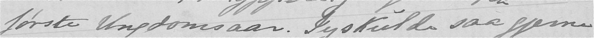første Ungdomsaar. Jeg skulde saa gjerne
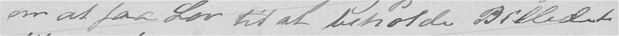om at faa Lov til at beholde Billedet
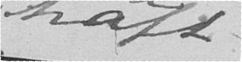haft
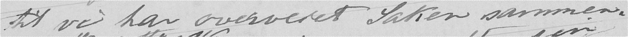til vi har overveiet Saken sammen.
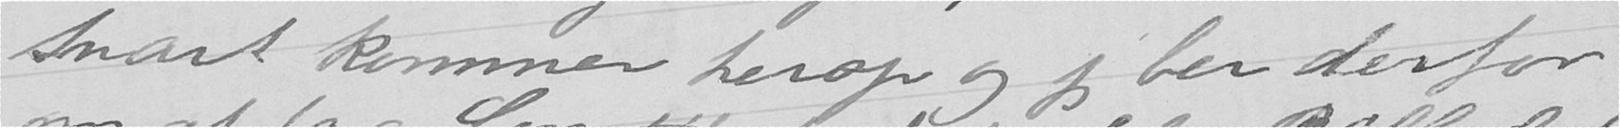snart kommer herop og jeg ber derfor
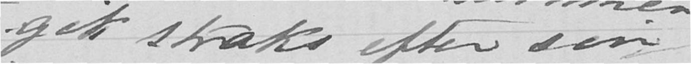gik straks efter sin
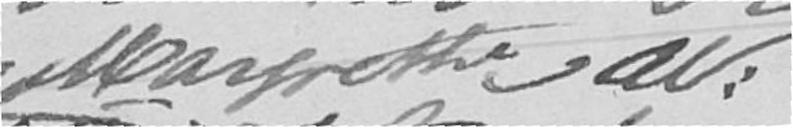Margrethe W.
